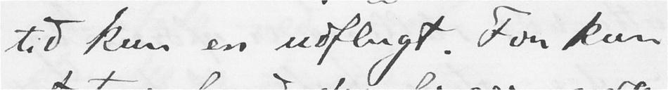tid kun en udflugt. For kun
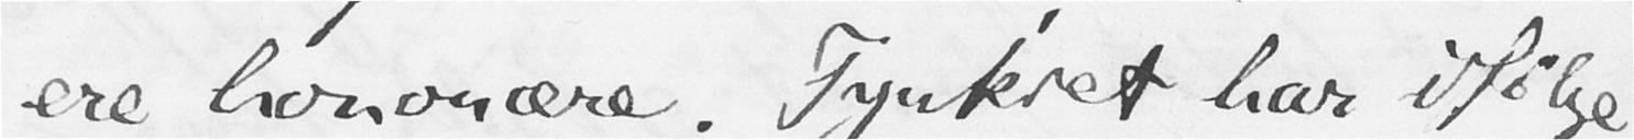ere honorære. Tyrkiet har ifølge
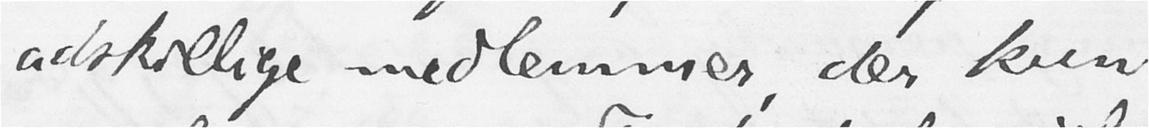adskillige medlemmer, der kun
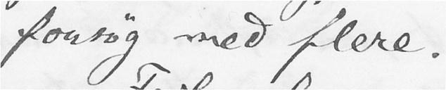forsøg med flere.
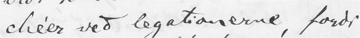chéer ved legationerne, fordi
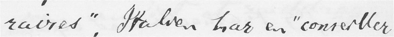raires", Italien har en "conseiller
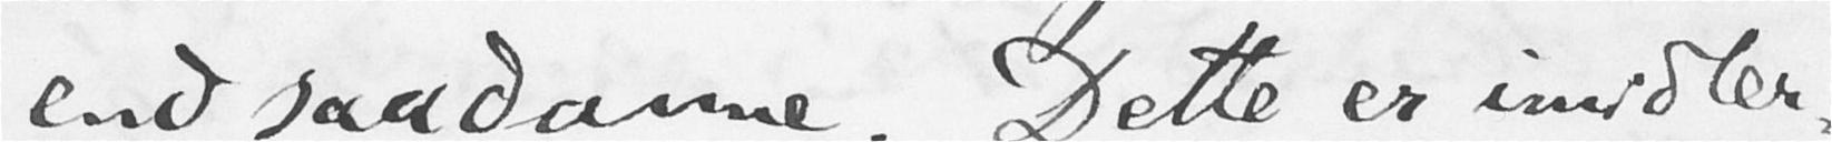end saadanne. Dette er imidler-
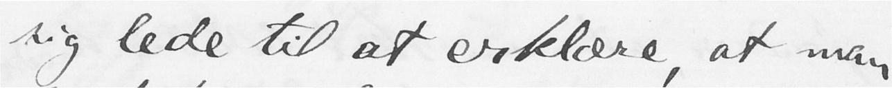sig lede til at erklære, at man
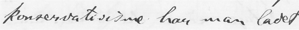konservativisme har man ladet
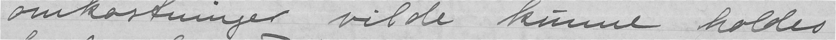omkostninger vilde kunne holdes
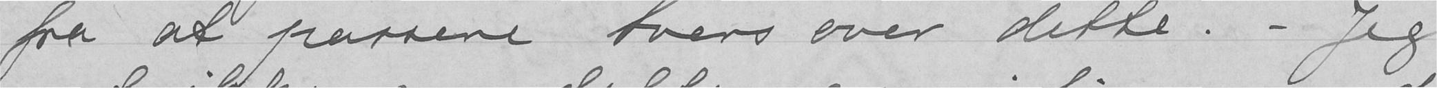fra at passere tvers over dette. - Jeg
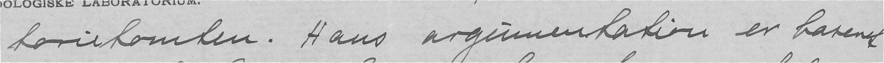torietomten. Hans argumentation er baseret
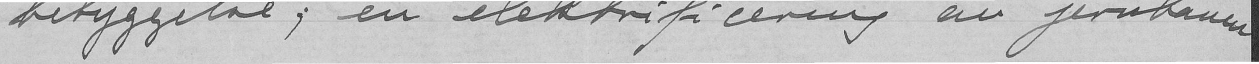bebyggelse; en elektrificering av jernbanen
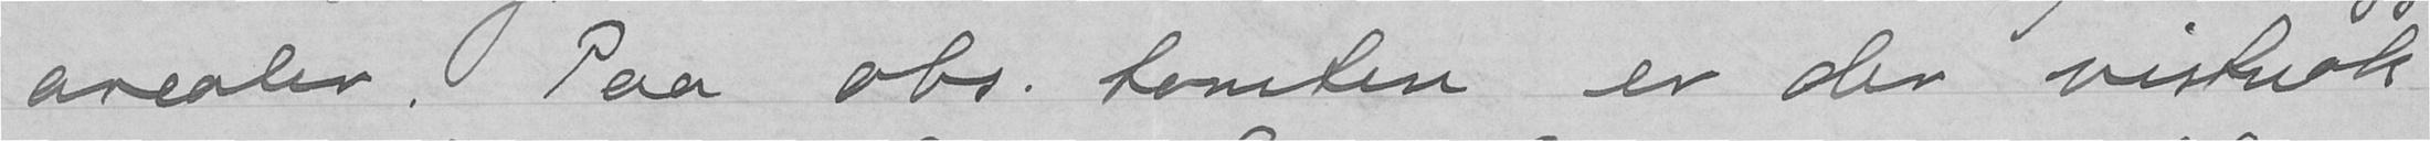arealer. Paa obs. tomten er der vistnok
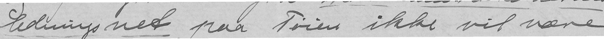ledningsnet paa Tøien ikke vil være
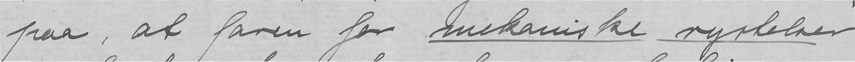paa, at faren for mekaniske rystelser
